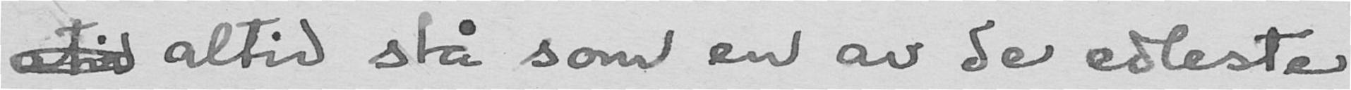atid altid stå som en av de edleste
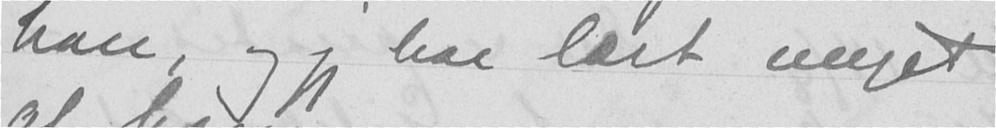han, og jeg har lært meget
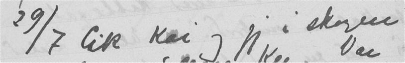29/7 Gik Kai og jeg i skogen
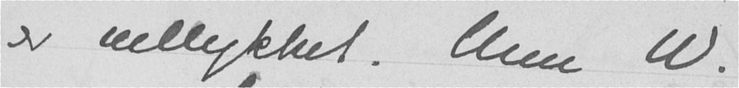er vellykket. Men W.
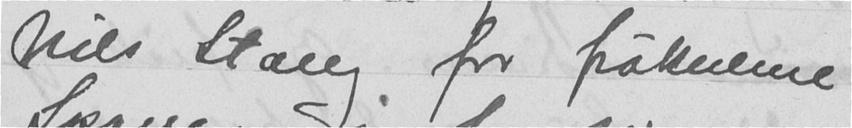Nils Stang for frøknene
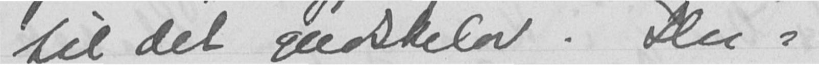til det gudskelov. Her-
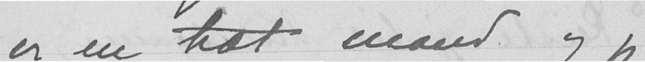er en træt mand og jeg
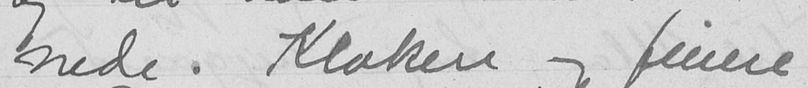nede. Klokere og finere
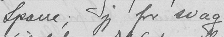Sparre; jeg for svag
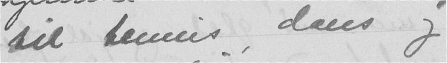til tennis, dans og
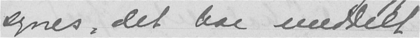synes, det har meddelt
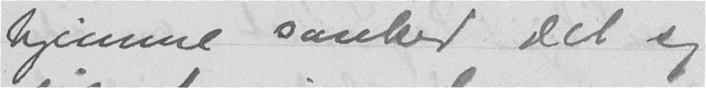hjemme sanked det sig
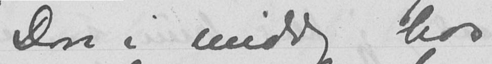Don i middag hos
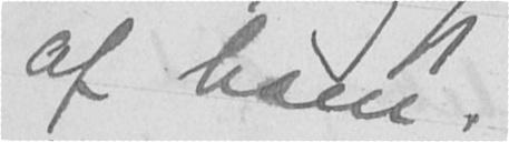af ham.
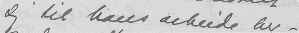sig til hans arbeide her-
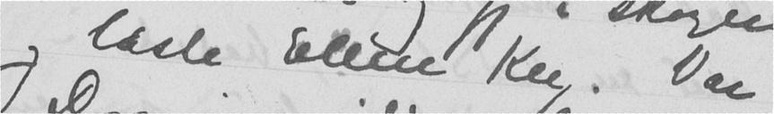og læste Ellen Key. Var
